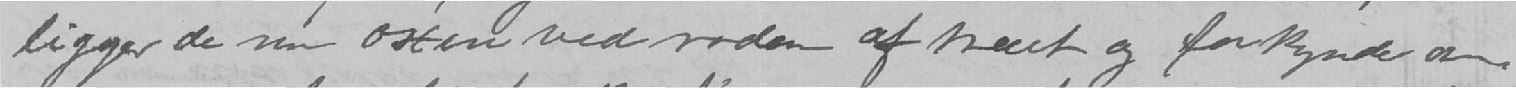ligger de nu oxen ved roden af træet og forkynder om-
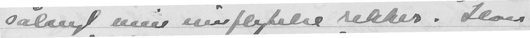sålangt min innflytelse rekker. Han
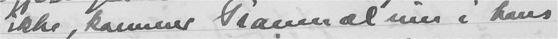ikke, kommer Frannal inn i hans
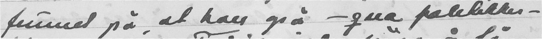funnet på, at han også - qua politiker -
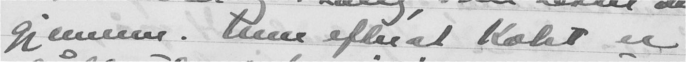gjennom. Den efterat Koht er
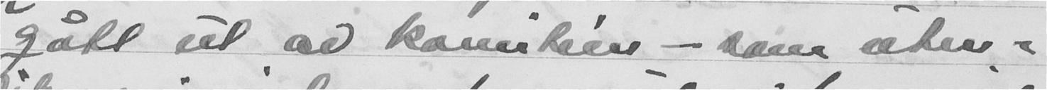gått ut av komitéen - som uten-
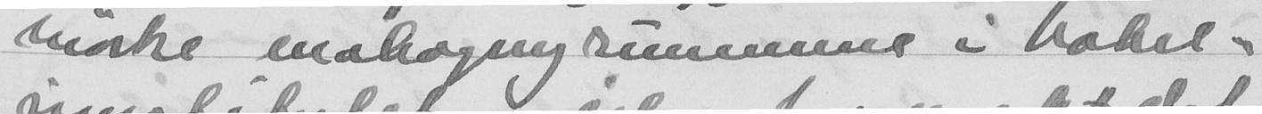mørke mahøygang rummene i bokel-
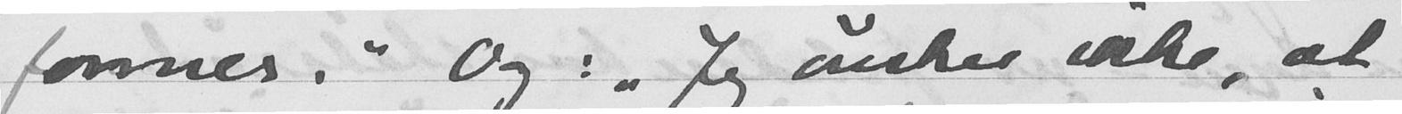former." Og: "Jeg ønsker ikke, at
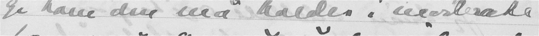gi ham den må holdes i moderate
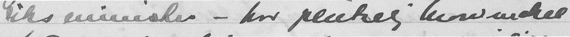riksminister - har plutselig Mowinckel
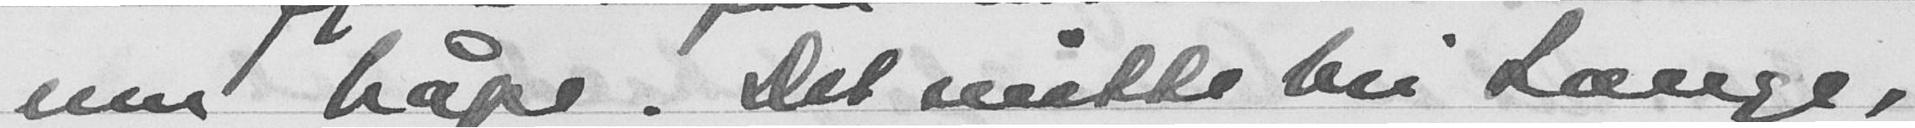enn håpe. Det måtte bli Lænze,
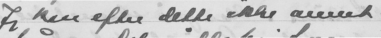Jeg kan efter dette ikke annet
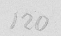120
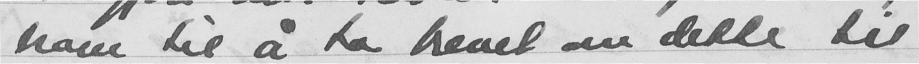ham til å ta brevet om dette til
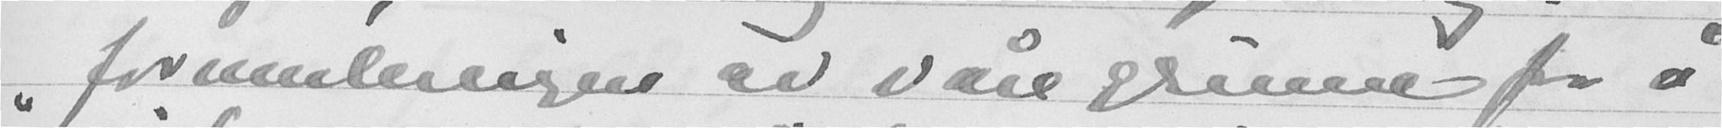"formuleringen av våre grunner for å
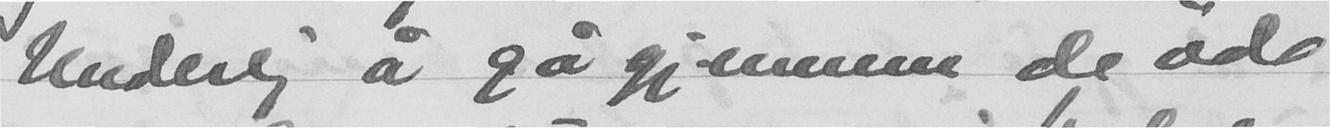Underlig å gå gjennem de øde
